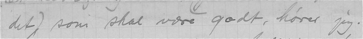det), som skal være godt, hører jeg.
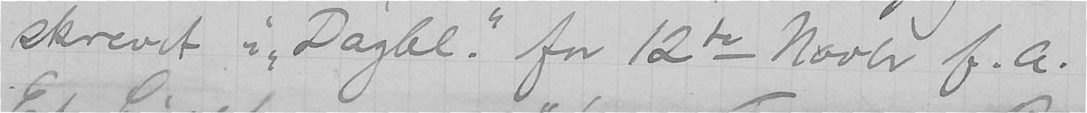skrevet i "Dagbl." for 12te Novbr f.A.
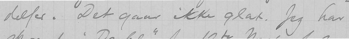delser. Det gaar ikke glat. Jeg har
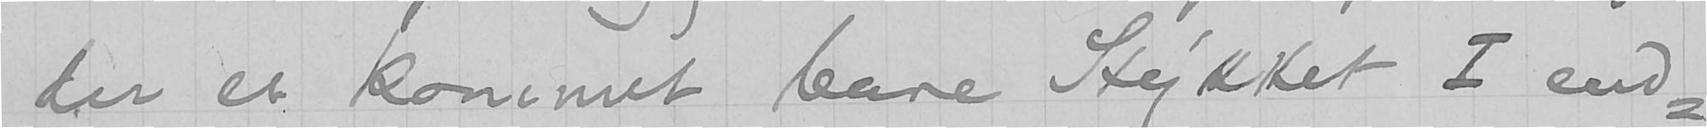der er kommet bare Stykket I end-
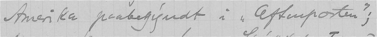Amerika paabegyndt i "Aftenposten";
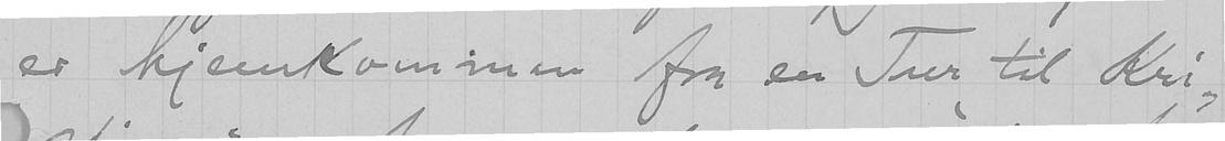er hjemkommen fra en Tur til Kri-
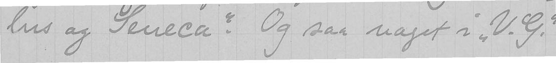lus og Seneca". Og saa noget i "V.G."
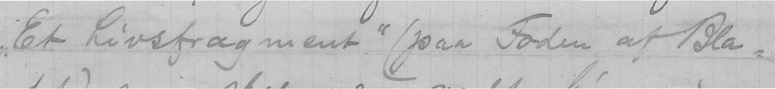Et Livsfragment " (paa Foden af Bla-
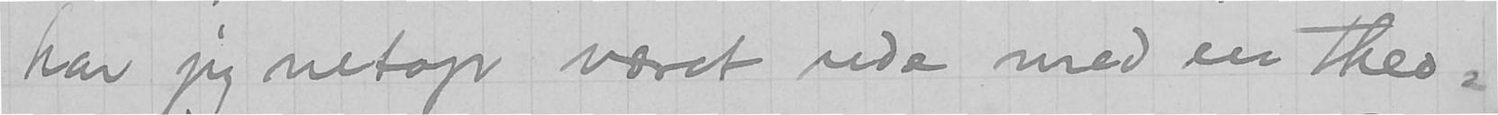har jeg netop været ude med en Theo-
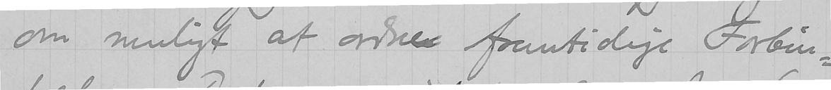om muligt at ordne fremtidige Forbin-
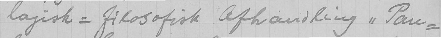logisk-filosofisk Afhandling "Pau-
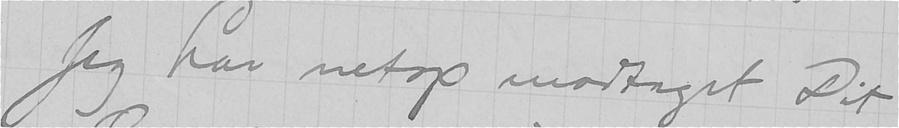Jeg har netop modtaget Dit
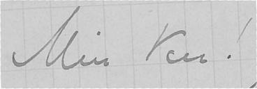Min Ven!
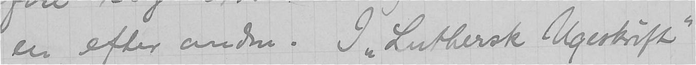en efter andre. I "Luthersk Ugeskrift"
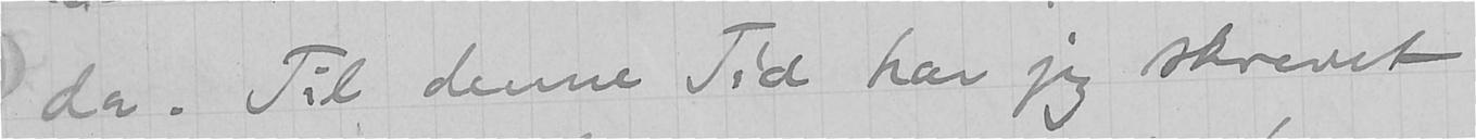da. Til denne Tid har jeg skrevet
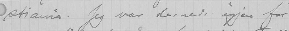stiania. Jeg var dernede igjen for
